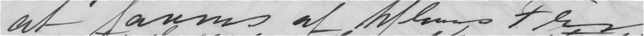at favnes af Aftnens Fred
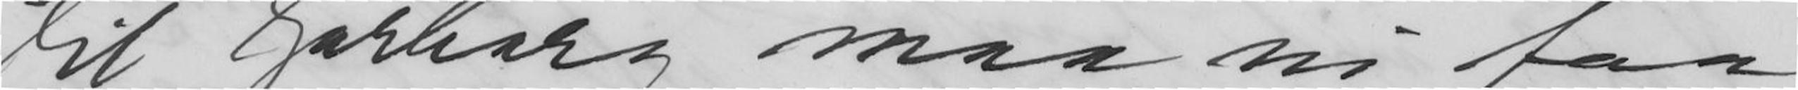til Garborg maa vi faa
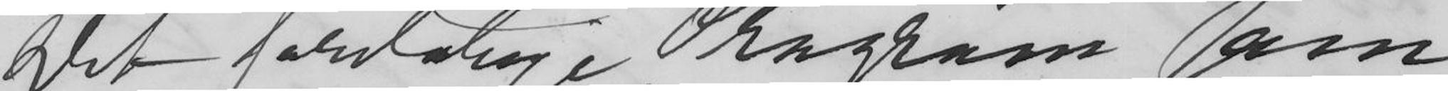Det foreløbige Program som
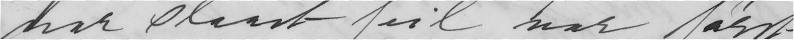var slaaet feil var først
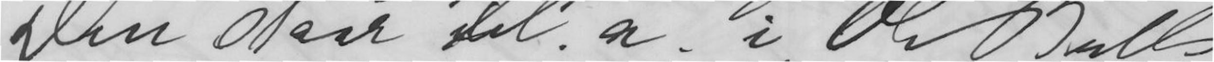(Den staar bl. a. i Ole Bulls
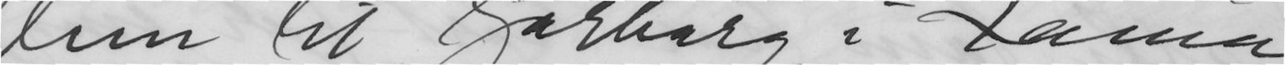Dem til Garborg i Xania
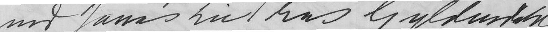ved Jonas Lie hos Gyldendahl)
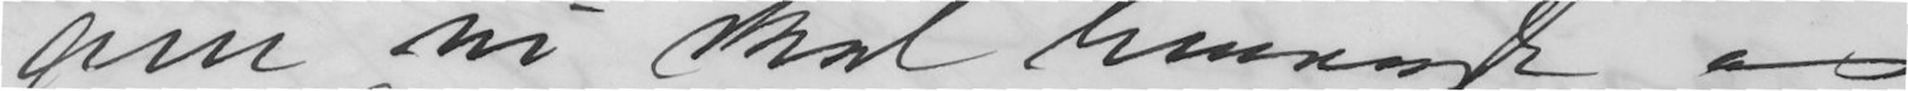om vi skal henvende os
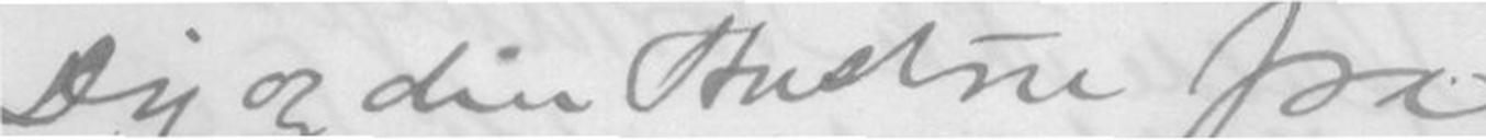Dig og din Hustru fra
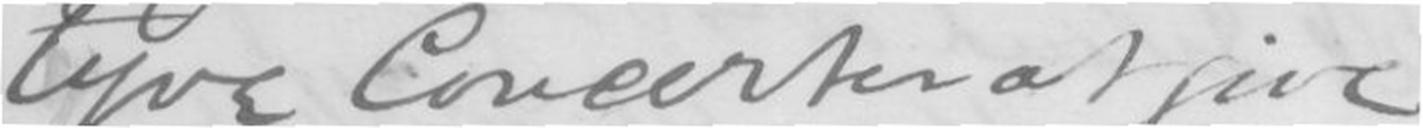tyve Concerter at give
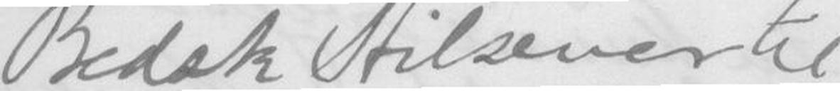Bedste Hilsener til
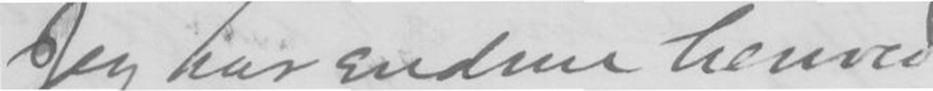Jeg har endnu hermed
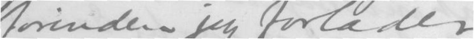forinden jeg forlader
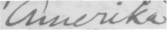Amerika
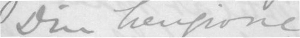Din hengivne
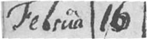Februa 16
